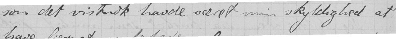som det vistnok havde været min skyldighed at
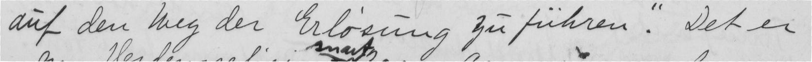auf den Weg der Erlósung yu führen". Det er
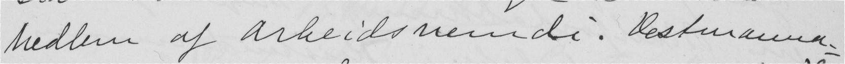Medlem af arbeidsnemdi. Vestmanna-
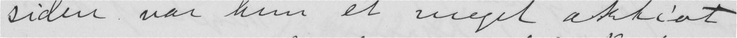siden var hun et meget aktivt
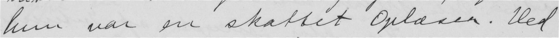hun var en skattet Oplæser. Ved
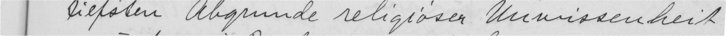tiefsten Abgrunde religióser Unmissenheit
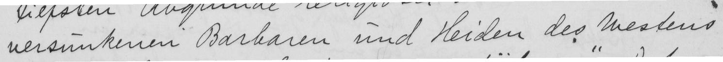versunkenen Barbaren und Heiden des Westens
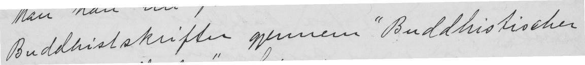Buddhistskrifter gjennem "Buddhistischer
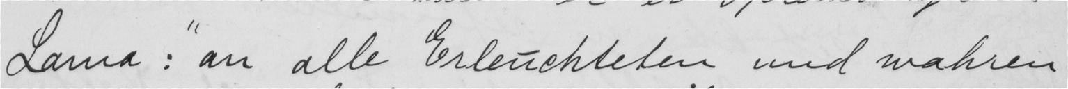Lama: "an alle Erleuchteten med wahren
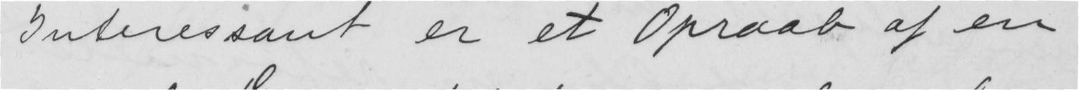Interessant er et Opraab af en
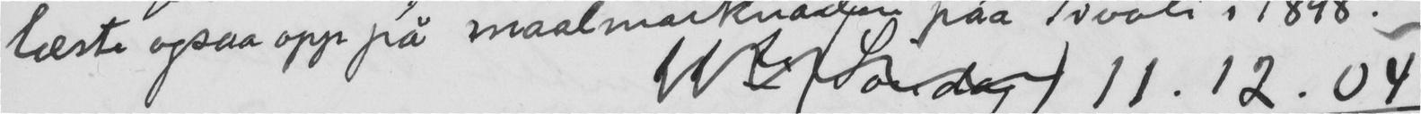læste ogsaa opp på maalmarknaden paa Tivoli i 1898.
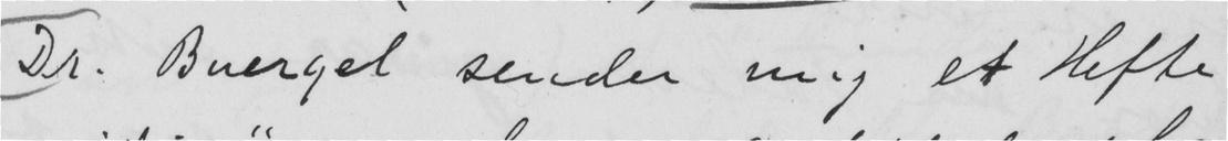Dr. Buergel sender mig et Hifte
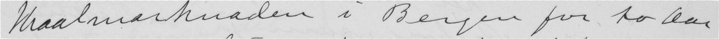Maalmarknaden i Bergen for to Aar
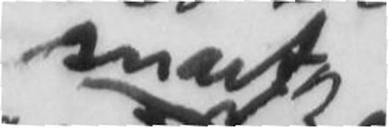snart
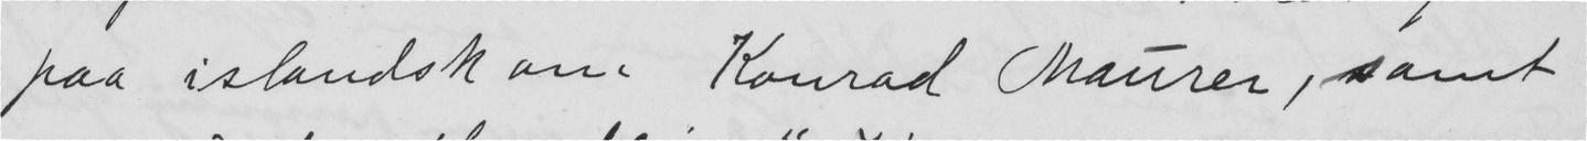paa islandsk om Konad Maurer, samt
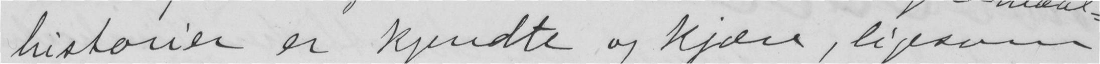historier er kjendte og kjære, ligesom
